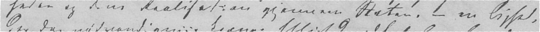heden og dens Realisation gjennem Staten. ¬ en Lighed,
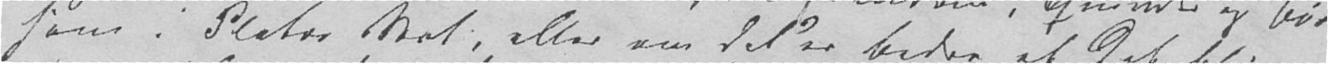som i Platos Stat, eller om det er bedre at det bliver
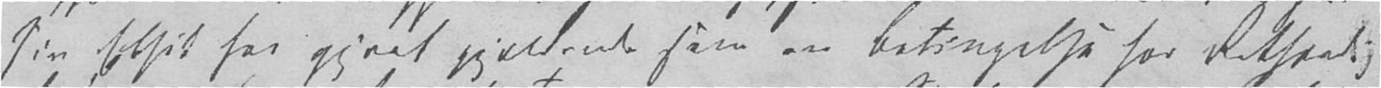sin Ethik har gjort gjældende som en Betingelse for Retfærdig-
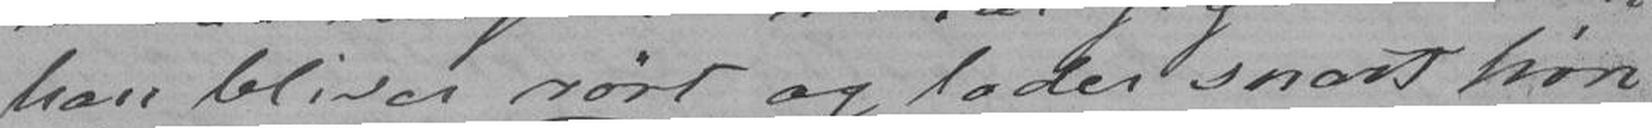han bliver rørt og lader snart høre
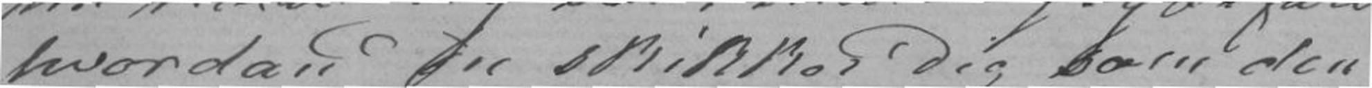hvordan Du skikker Dig som den
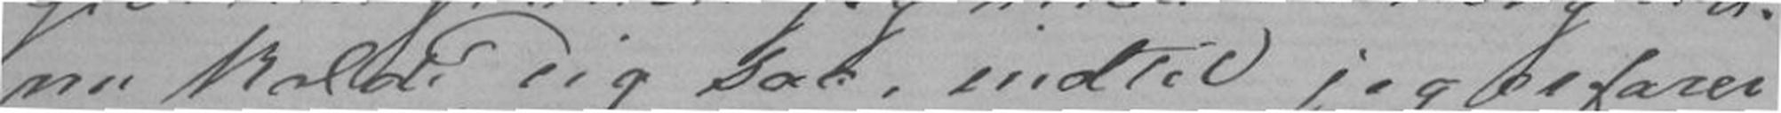nu kalde Dig saa, indtil jeg erfarer
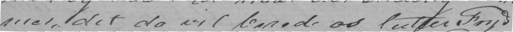mer, det da vil berede os lutter Fryd
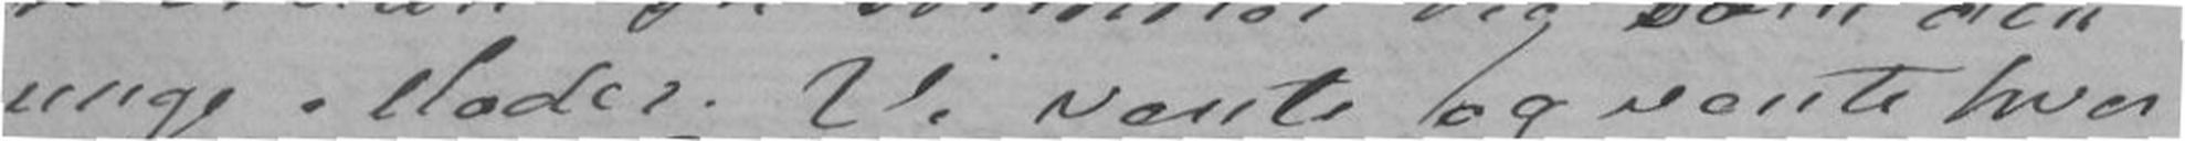unge Moder. Vi vænte og vænte hver
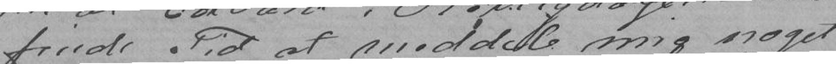finde Tid at meddele mig noget
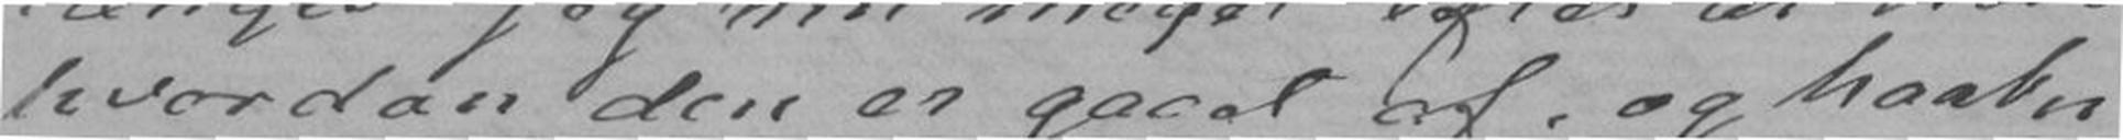hvordan den er gaaet af, og haaber
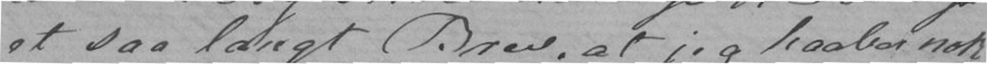et saa langt Brev, at jeg haaber nok
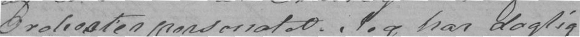Orchesterpersonalet. Jeg har daglig
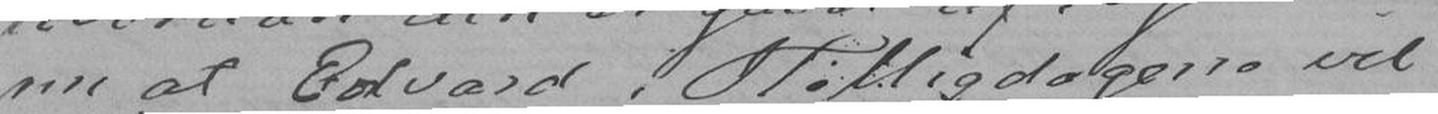nu at Edvard i Helligdagene vil
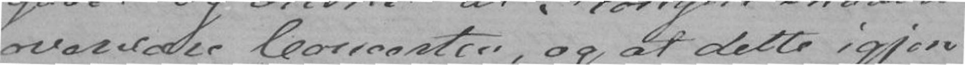overvære Concerten, og at dette igjen
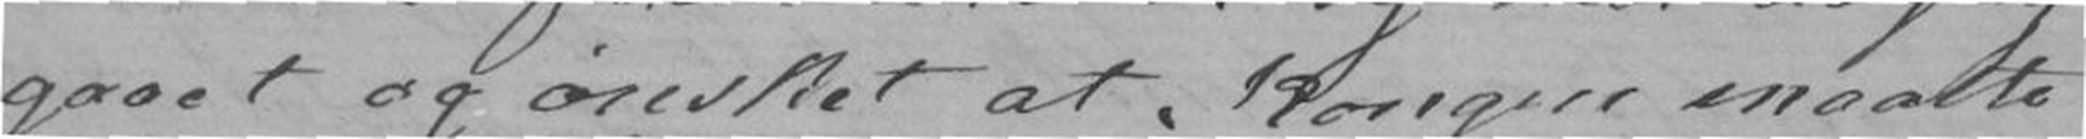gaaet og ønsket at Kongen maatte
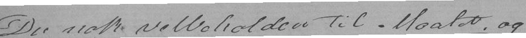Du nok velbeholden til Maalet, og
